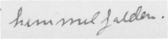himmelfalden.
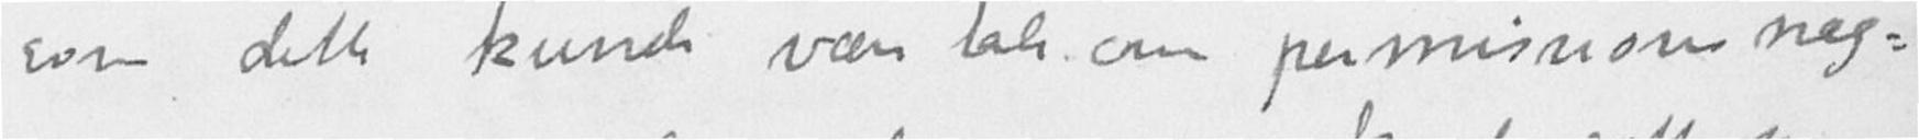som dette kunde være tale om permissionsnæg-
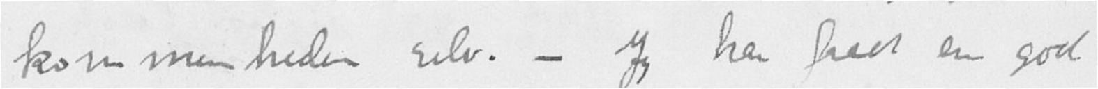kommenheden selv. - Jeg har faaet en god
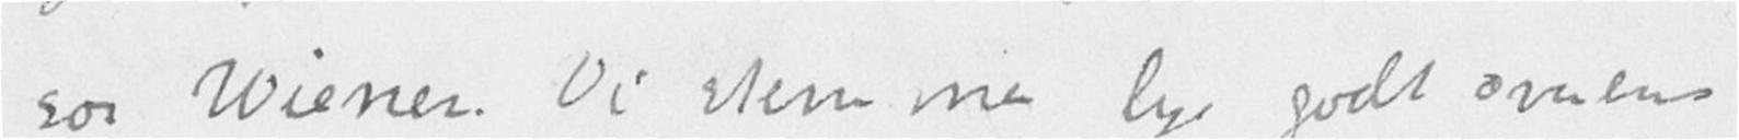sor Wiener. Vi stemmer lige godt overens
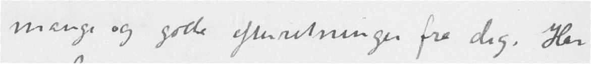mange og gode efterretninger fra dig. Har
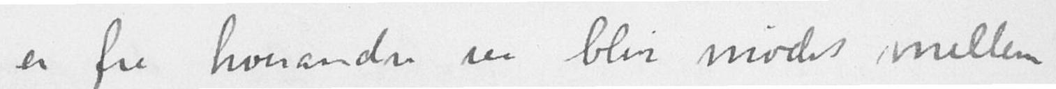er fra hverandre saa blir mødet mellem
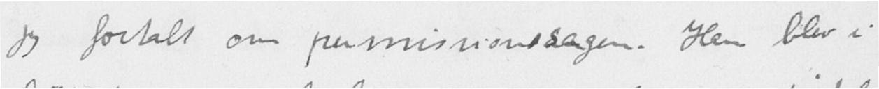jeg fortalt om permissionssagen. Han blev i
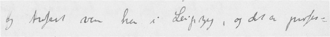og trofast ven her i Leipzig, og det er profes-
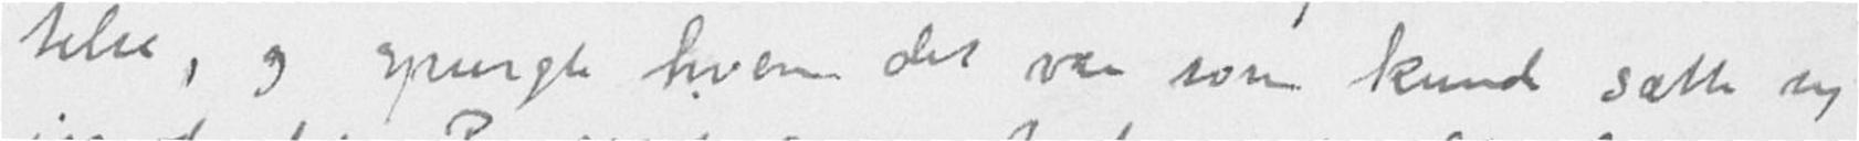telse, og spurgte hvem det var som kunde sætte sig
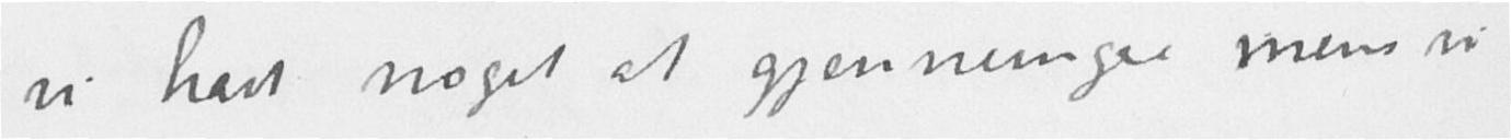vi havt noget at gjennemgaa mens vi
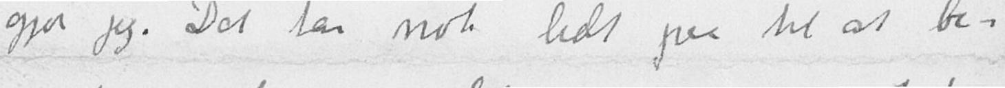gjør jeg. Det tar nok lidt paa til at be-
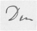Din
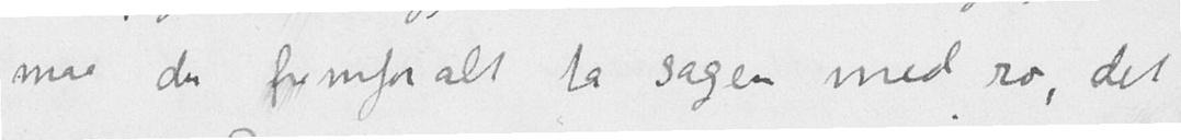maa du fremfor alt ta sagen med ro, det
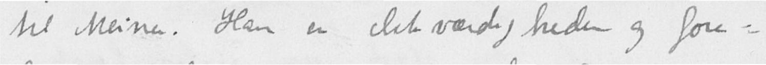til Meiner. Han er elskværdigheden og fore-
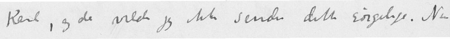Karl, og da vilde jeg ikke sende dette sørgelige. Nu
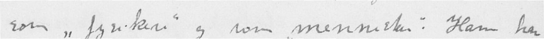som "fysikere" og som "mennesker". Ham har
